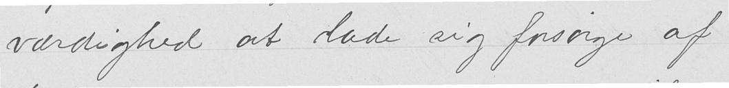værdighed at lade sig forsørge af
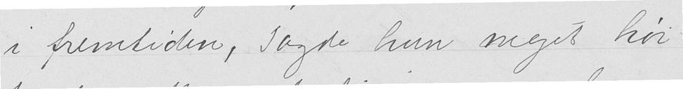i fremtiden, sagde hun meget høi-
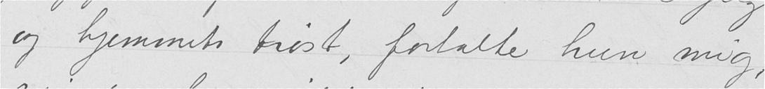og hjemmets trøst, fortalte hun mig,
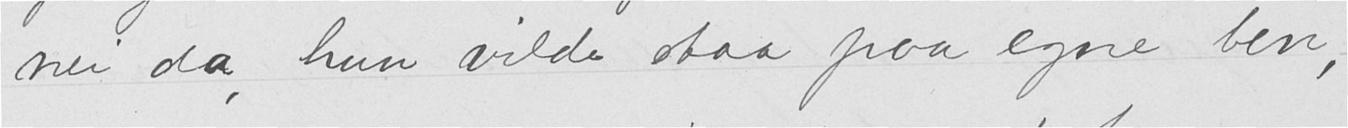nei da, hun vilde staa paa egne ben,
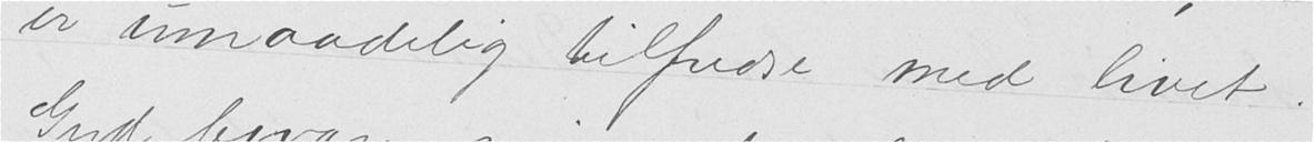er umaadelig tilfredse med livet.
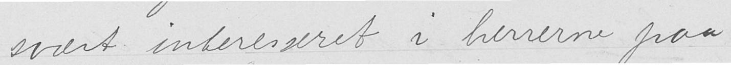svært interesseret i herrerne paa
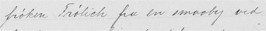frøken Frølich fra en smaaby ved
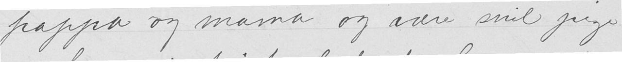pappa og mama og være snil pige
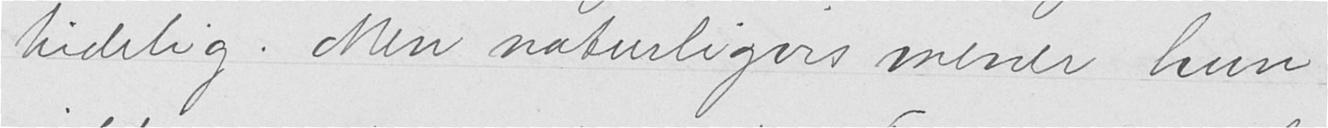tidelig. Men naturligvis mener hun
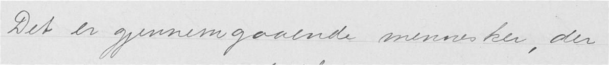Det er gjennemgaaende mennesker, der
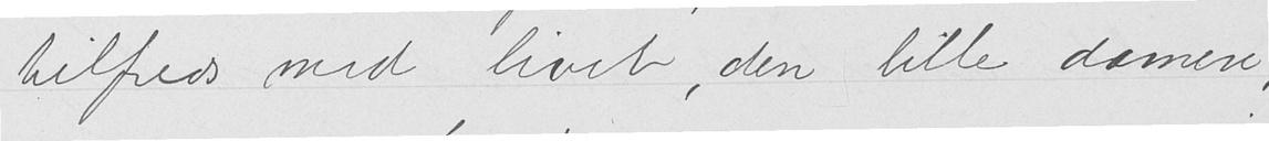tilfreds med livet, den lille damen,
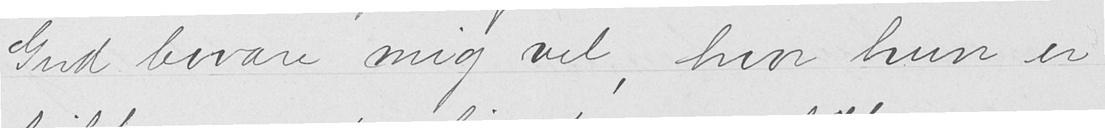Gud bevare mig vel, hvor hun er
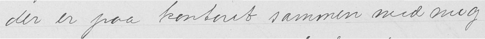der er paa kontoret sammen med mig.
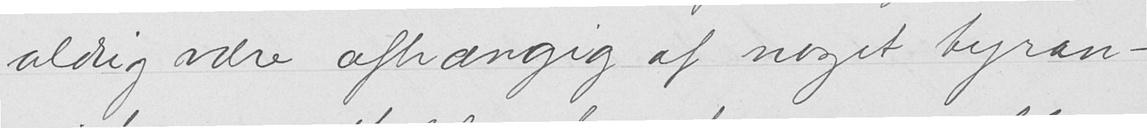aldrig være afhængig af noget tyran-
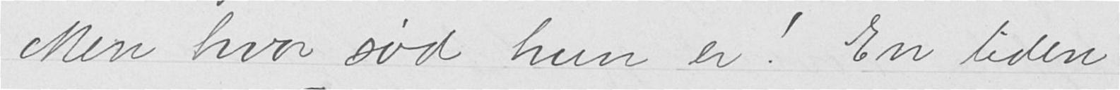Men hvor sød hun er! En liden
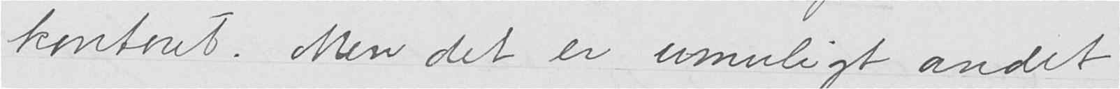kontoret. Men det er umuligt andet
